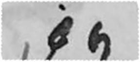jeg
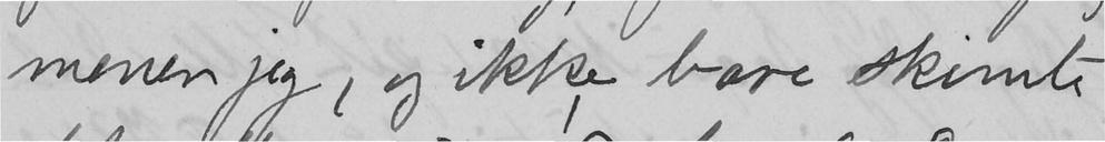mener jeg, og ikke bare skimte
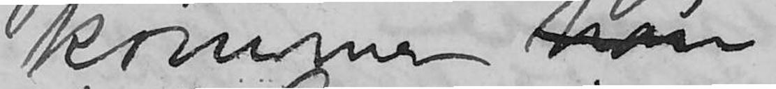kommer han
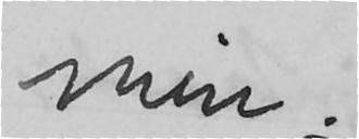min.
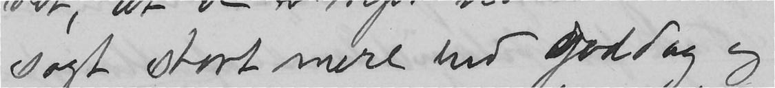sagt stort mere end goddag og
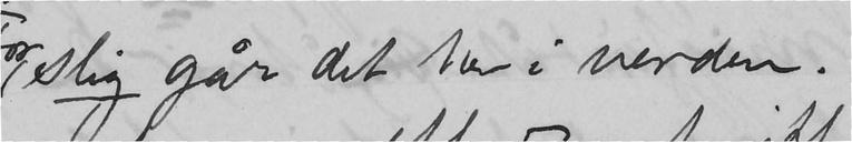slig går det her i verden.
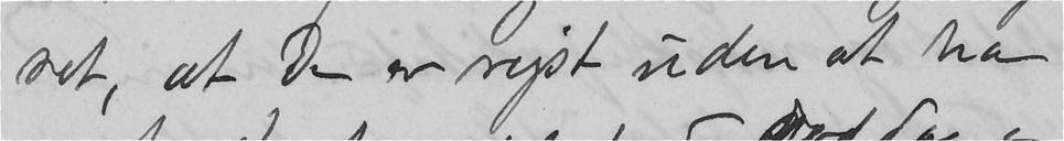ret, at Du er rejst uden at ha
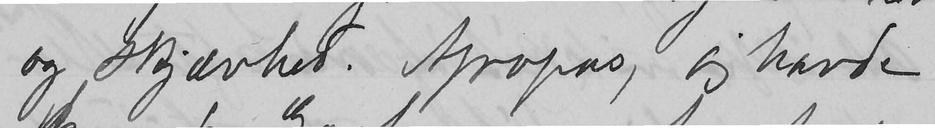og skjævhed. Apropos, jeg havde
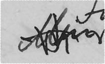Men
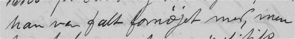han var fælt fornøjet med, men
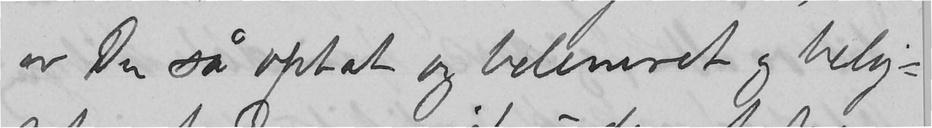er Du så optat og belemret og belej-
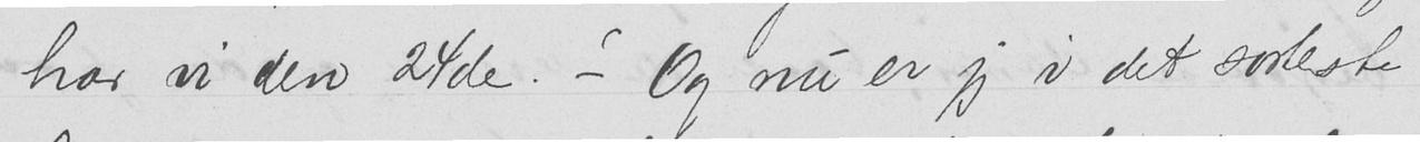har vi den 24de. - Og nu er jeg i det sorteste
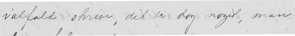ialfald skrive, det er dog noget, man
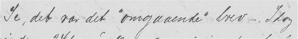Se, det var det "omgaaende" brev -. Idag
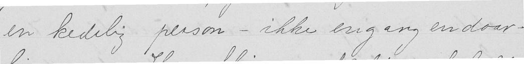en kedelig person - ikke engang en daar-
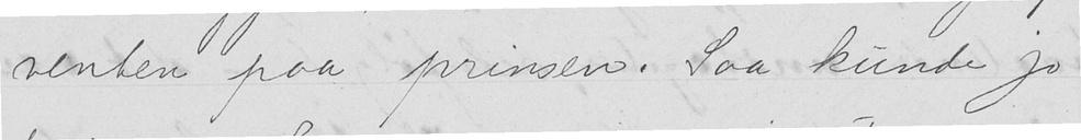venten paa prinsen. Saa kunde jo
